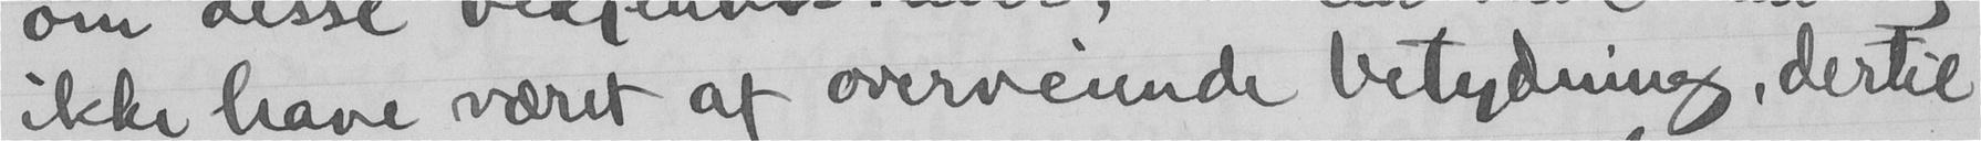ikke have været af overveiende betydning, dertil
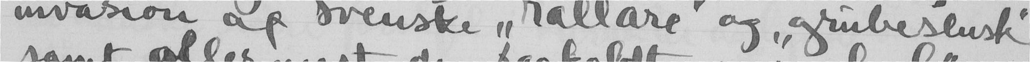invasion af svenske "rallare" og "grubeselusk"
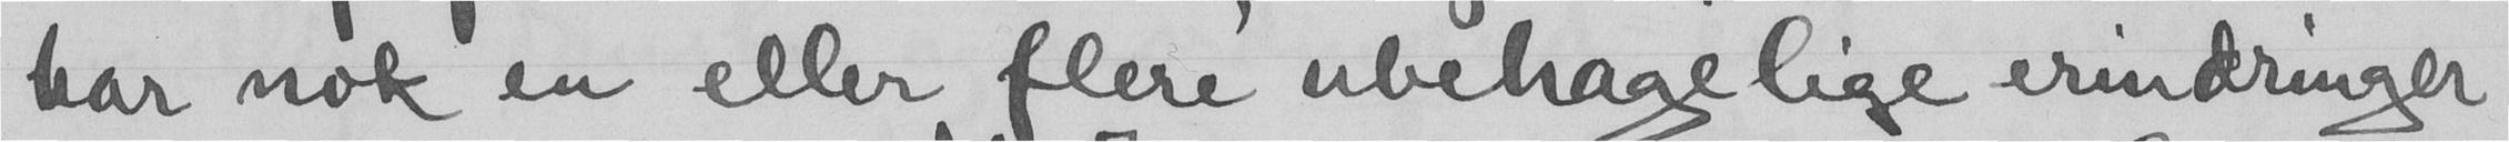har nok en eller flere ubehagelige erindringer
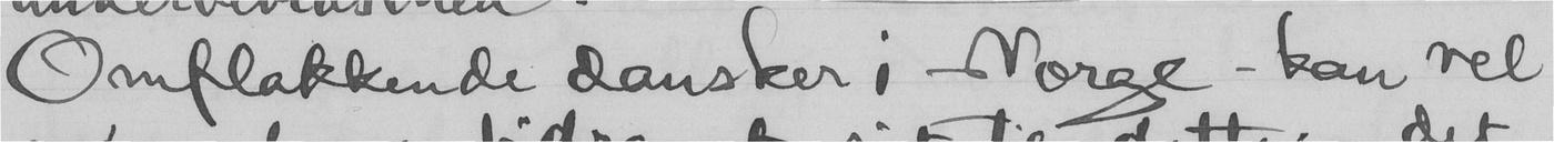Omflakkende dansker i Norge - kan vel
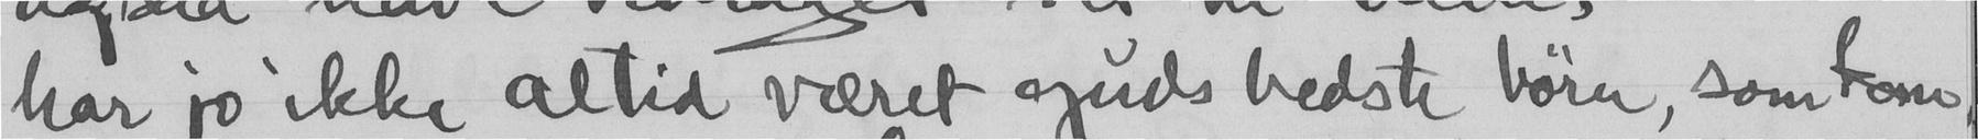har jo ikke altid været gguds bedste børn, som kom,
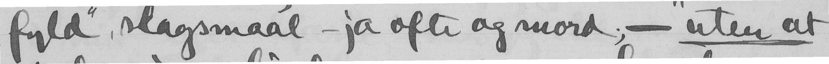"fyld", slagsmaal - ja ofte og mord; - uten at
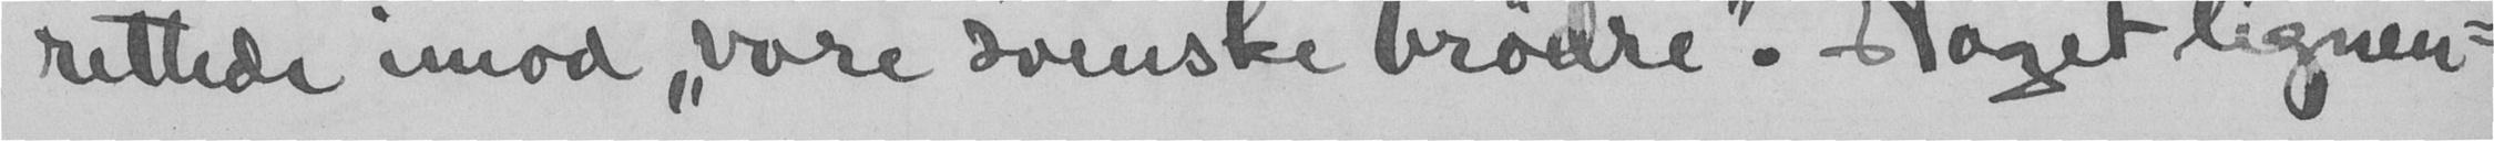rittede imod "vore svenske brødre". Noget lignen-
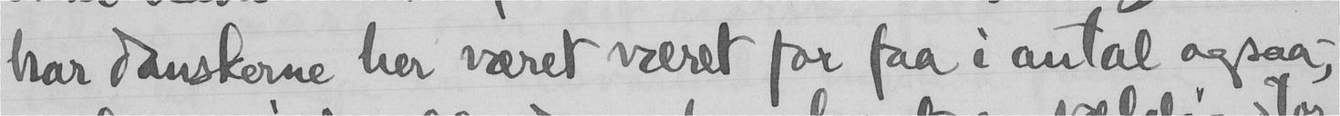har danskerne her været været for faa i antal ogsaa,
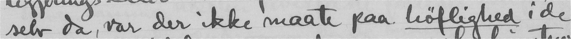selv da, var der ikke maate paa høflighed i de
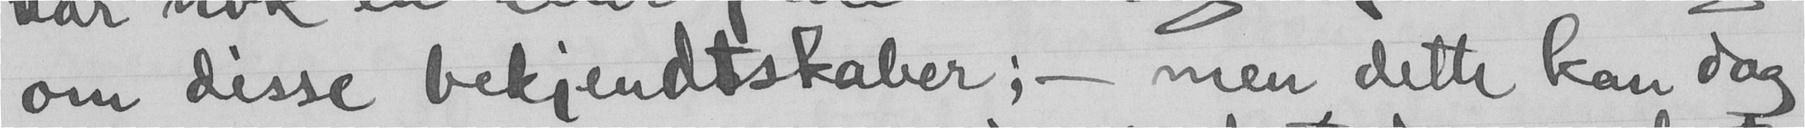om disse bekjendtskaber; - men dette kan dog
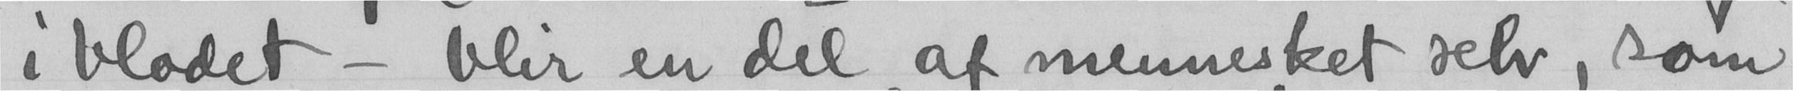i blodet - blir en del af mennesket selv, som
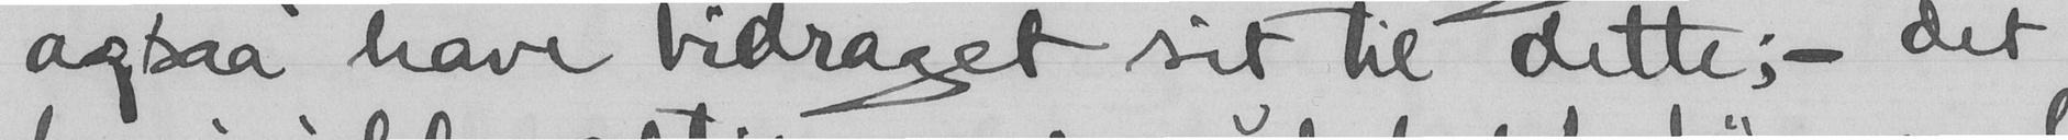ogsaa have bidraget sit til dette, - det
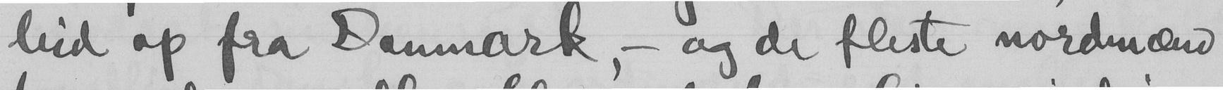hid op fra Dannark - og de fleste nordmænd
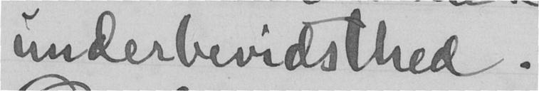underbevidsthed.
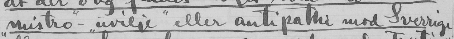"mistro"-"uvilje" eller antipathi mod - Sverrige
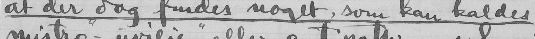at der dog findes noget, som kan kaldes
